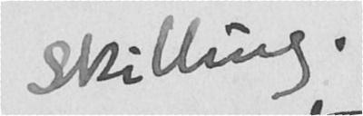skilling.
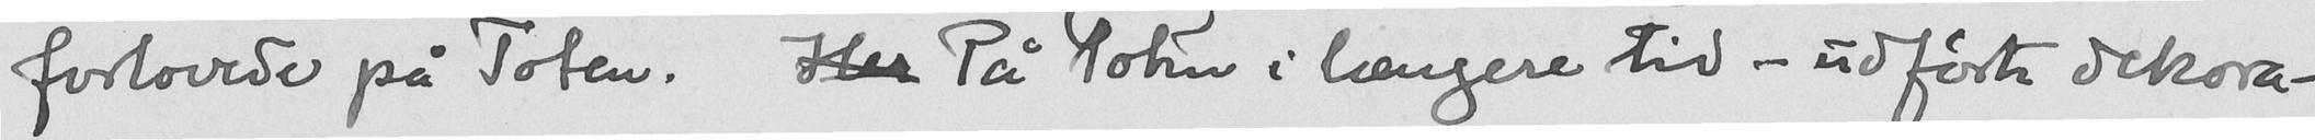forlovede på Toten. Her På Toten i længere tid - udførte dekora-
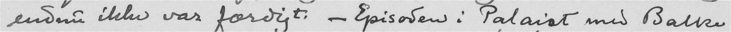endnu ikke var færdigt. - Episoden i Palaist med Balke
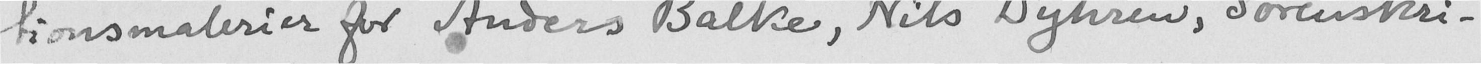tionsmalerier for Anders Balke, Nils Dyhren, sorenskri-
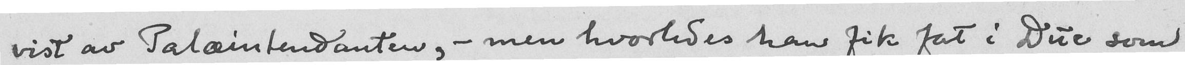vist av Palæintendanten, - men hvorledes han fik fat i Due som
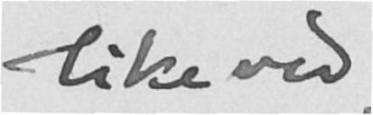like ved
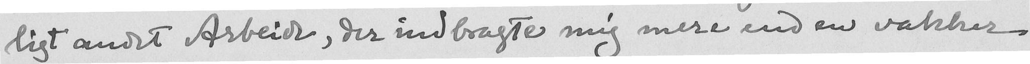ligt andet Arbeide, der indbragte mig mere end en vakker
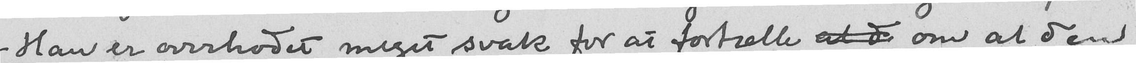- Han er overhodet meget svak for at fortælle al d om al den
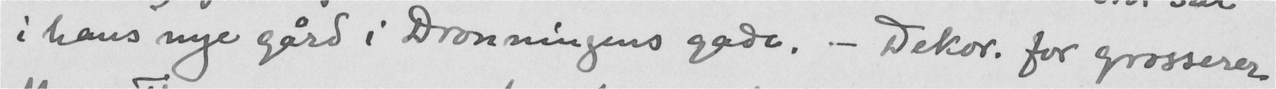i hans nye gård i Dronningens gade. - Dekor. for grosserer
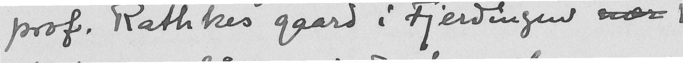prof. Rathkes gaard i Fjerdingen nær
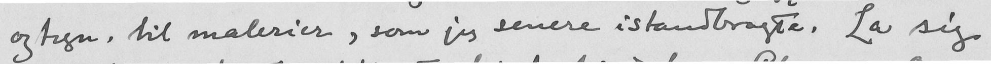og tegn, til malerier, som jeg senere istandbragte. La sig
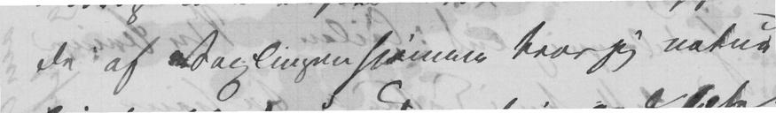de af Vexlingen hjemme tror jeg natur
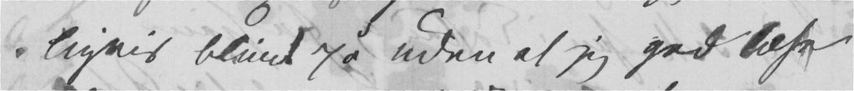ligvis blindt på uden at jeg gad læse
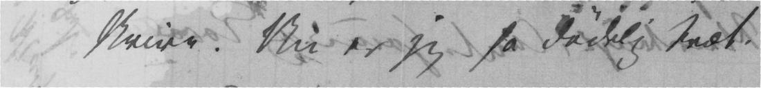skrive. Nu er jeg sa dødelig træt.
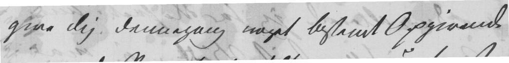give Dig dennegang noget bestemt Opgivende
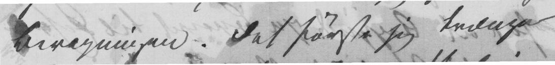Beregningen. Det første jeg trænger
